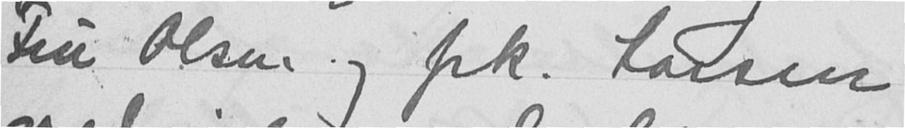Fru Olsen og frk. Larsen
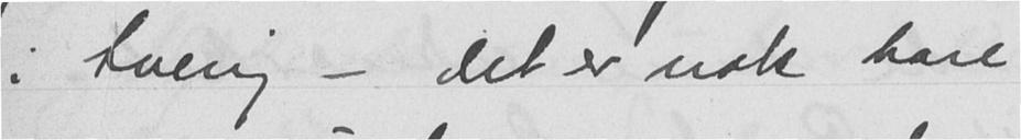i Sverig - det er nok bare
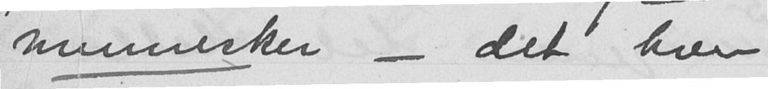mennesker - det hver
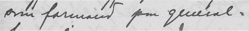som formand paa general-
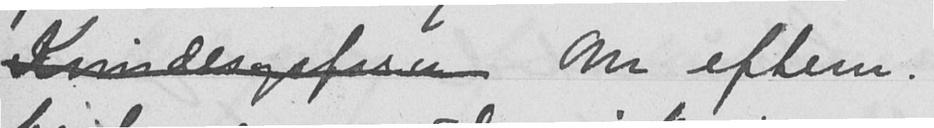Kvindesagsforn. Om efterm.
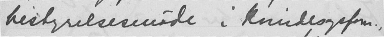bestyrelsesmøde i Kvindesagsforn.,
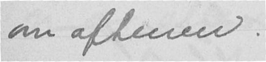om aftenen.
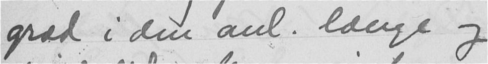græd i den anl. længe og
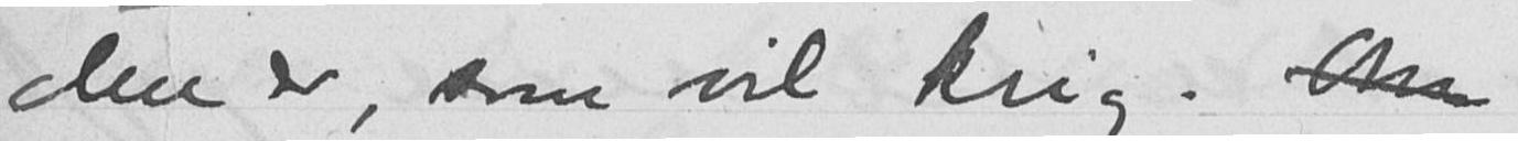den er, som vil krig. Om
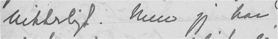bitterligt. Men jeg har
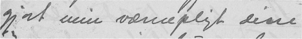gjort men værnepligt disse
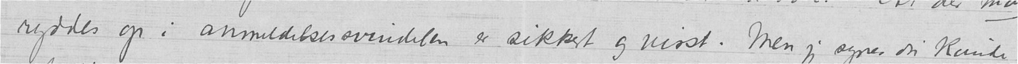ryddes op i anmeldelsessvindelen er sikkert og visst. Men jeg synes du kunde
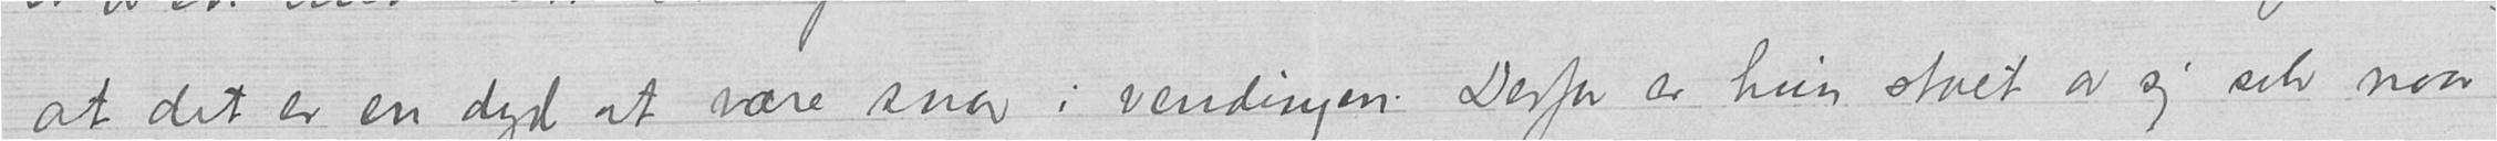at det er en dyd at være snar i vendingen. Derfor er hun stolt av sig selv naar
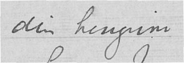din hengivne
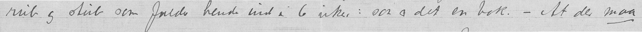rub og stub som falder hende ind i 6 uker: saa er det en bok. – At der maa
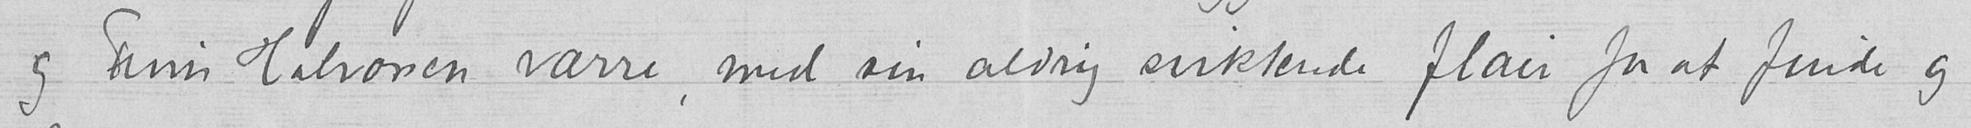og Finn Halvorsen værre, med sin aldrig sviktende flair for at finde og
